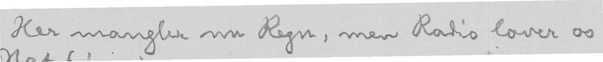Her mangler nu Regn, men Radio lover os
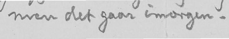men det gaar imorgen.
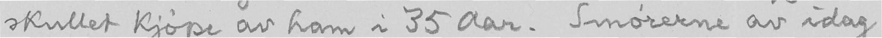skullet kjøpe av ham i 35 Aar. Smørerne av idag
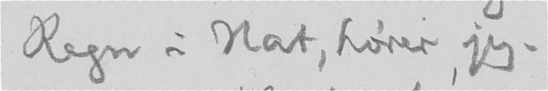Regn i Nat, hører jeg.
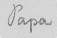Papa
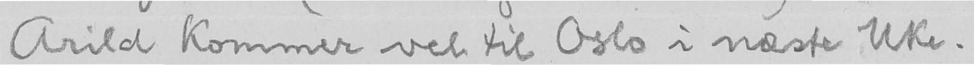Arild kommer vel til Oslo i næste Uke.
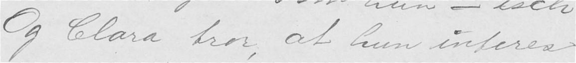Og Clara tror, at hun interes-
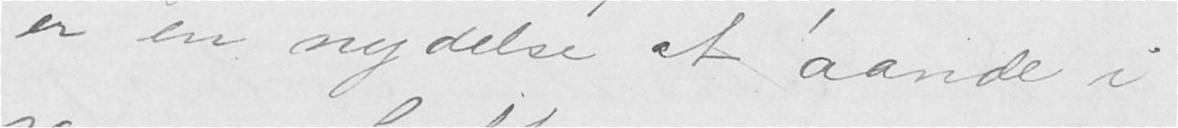er en nydelse at aande i
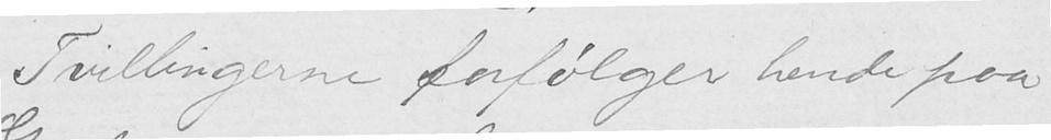Tvillingerne forfølger hende paa
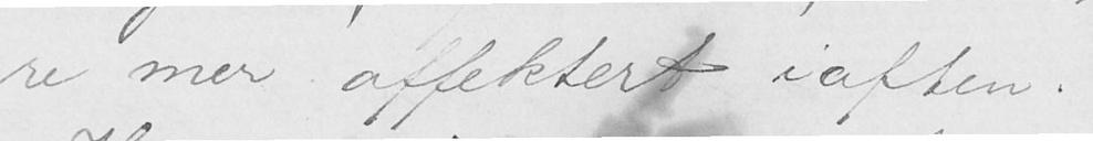re mer affektert iaften.
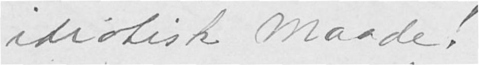idiotisk Maade!
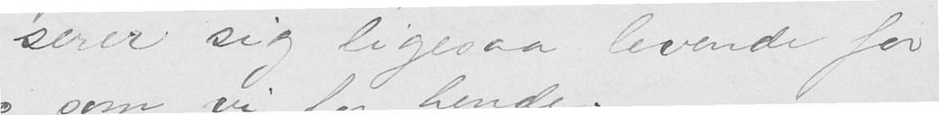serer sig ligesaa levende for
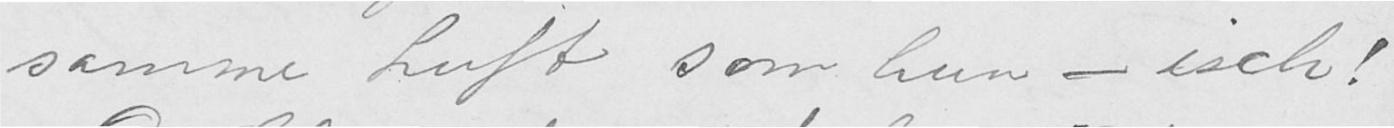samme Luft som hun — isch!
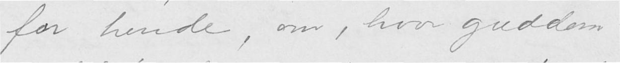for hende, om, hvor guddom-
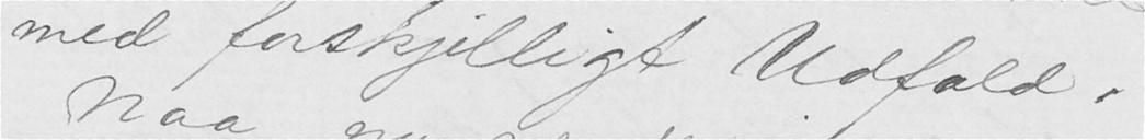med forskjelligt Udfald.
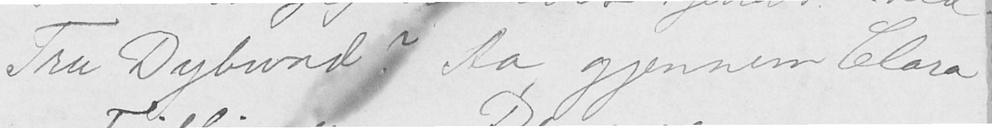Fru Dybwad? Aa, gjennem Clara
